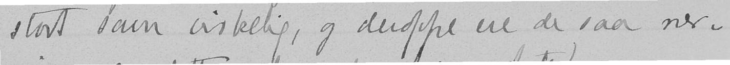stort savn virkelig, og deroppe ere de saa ner-
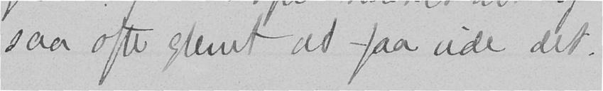saa ofte glemt at faa vide det.
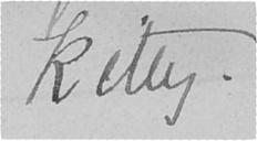Kitty.
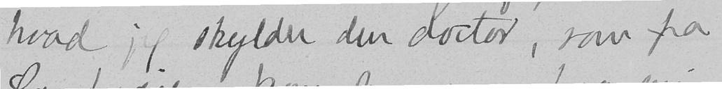hvad jeg skylder den doctor, som fra
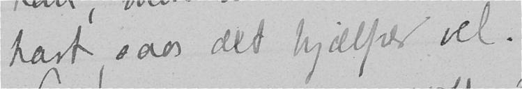hart, saa det hjælper vel.
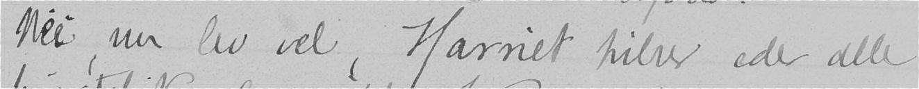Nei, nu lev vel, Harriet hilser eder alle
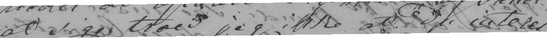at sige troer jeg ikke at Du interes-
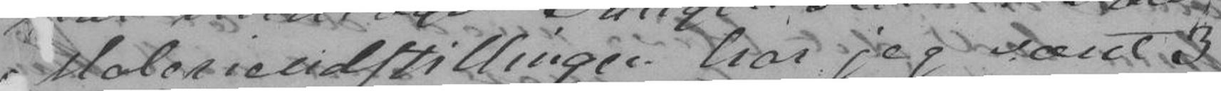Malerieudstillingen har jeg været 3
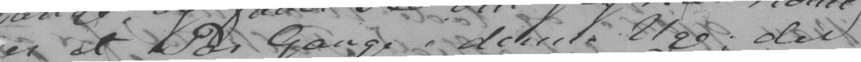er et Par Gange i denne Uge; der
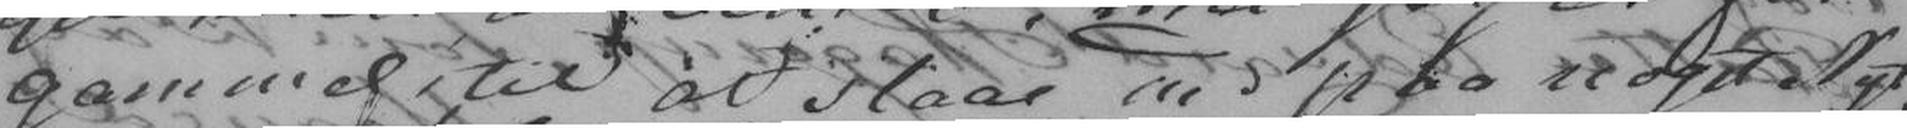gammel til at slaae ind paa noget Nyt,
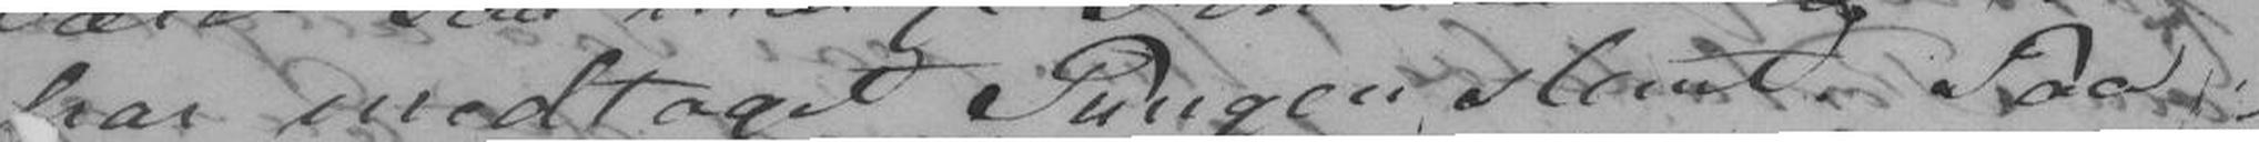har medtaget Pungen slemt. Paa
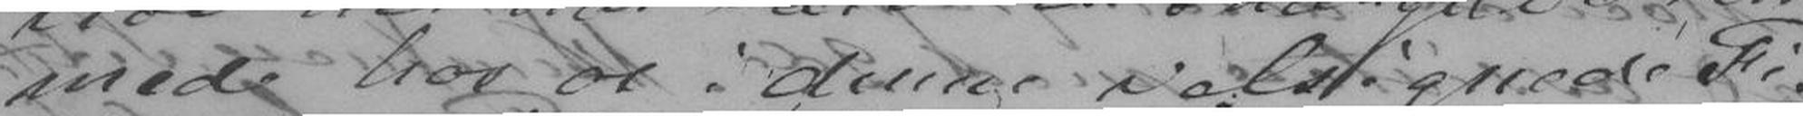mede hos os i denne velsignede Fi-
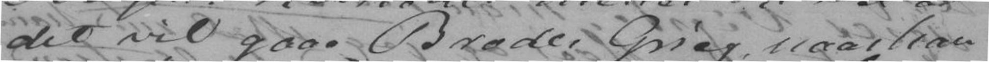det vil gaae Broder Grieg, naar han
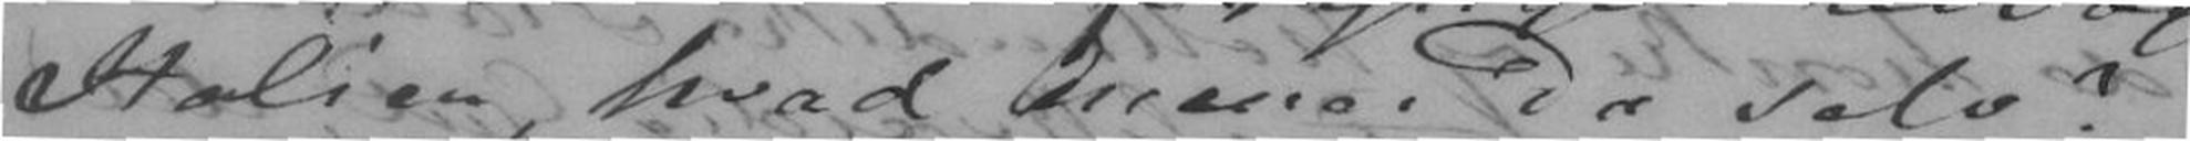Italien, hvad mener Du selv?
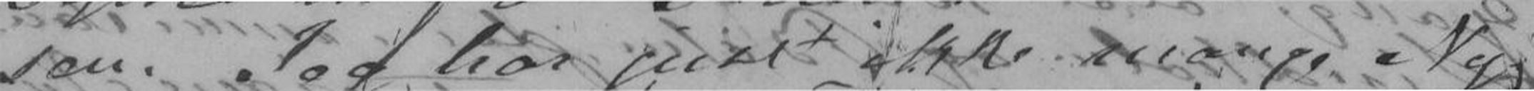sen. Jeg har just ikke mange Ny-
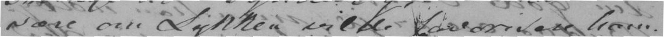være om Lykken vilde favorisere ham.
